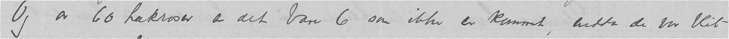Og av 60 høkroser er det bare 6 som ikke er kommet, endda de var blit
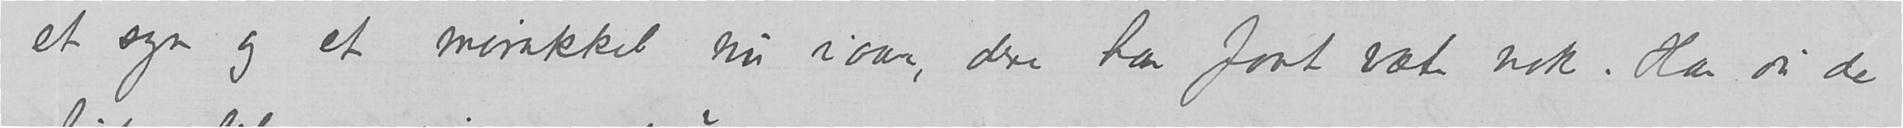et syn og et mirakkel nu iaar, dere har faat væte nok. Har du de
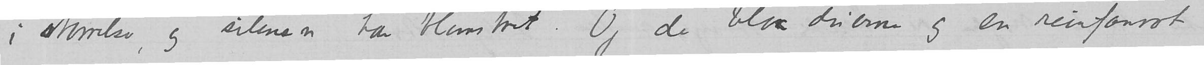i størrelser, og silenen har blomstret.  Og de blaa druerne og en reinfanrot
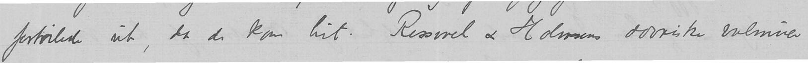fortvilede ut, da de kom hit. Ressvold & Holmsens dovriske valmuer
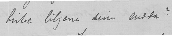hvite liljene dine endda?
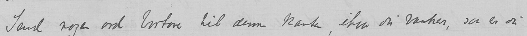Send nogen ord bortover til denne kanten, ihvor du vanker, saa er du
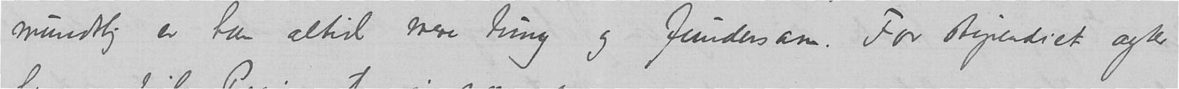mundtlig er han altid mere tung og fundersom. For stipendiet agter
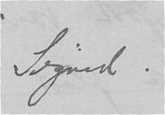Sigrid
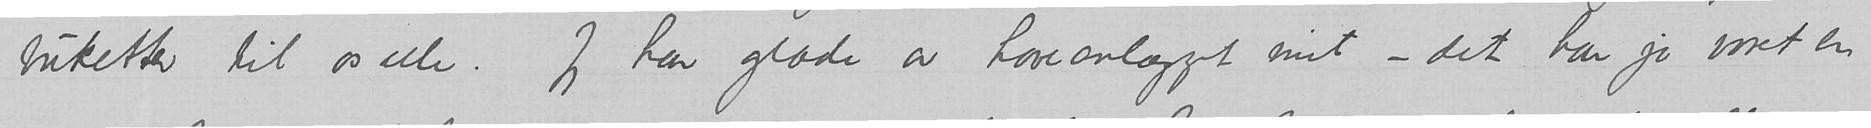buketter til os alle. Jeg har glæde av haveanlægget mit – det har jo været en
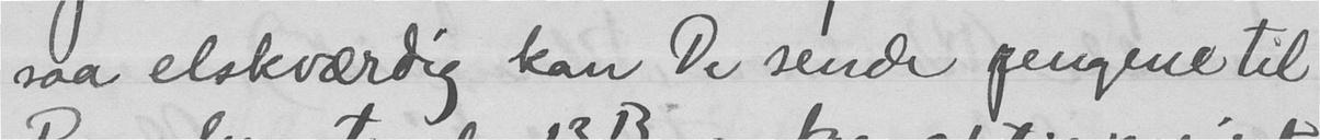saa elskværdig kan De sende pengene til
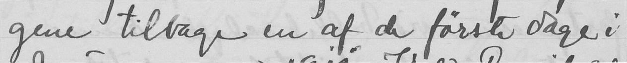gene tilbage en af de første dage i
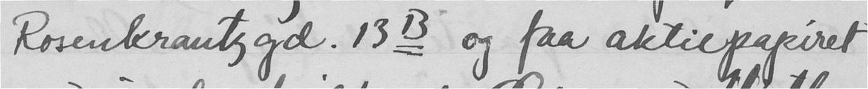Rosenkrantzgd. 13B og faa aktiepapiret
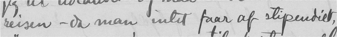reisen - da man intet faar af stipendiet,
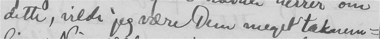dette, vilde jeg være Dem meget taknem-
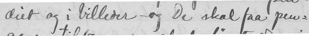diet og i billeder - og De skal faa pen-
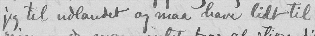jeg til udlandet og maa have lidt til
